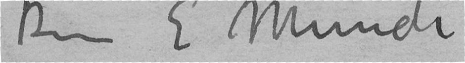Din E Munch
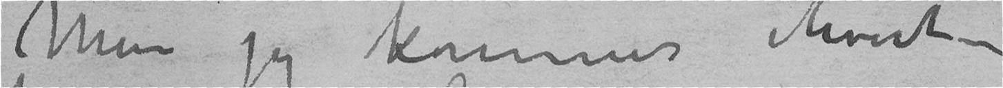Men jeg kommer ihvert-
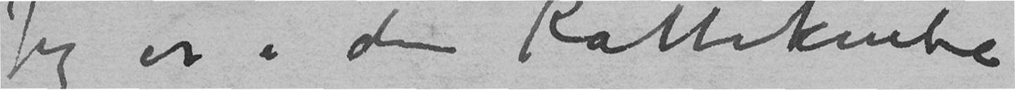Jeg er i den Katteknibe
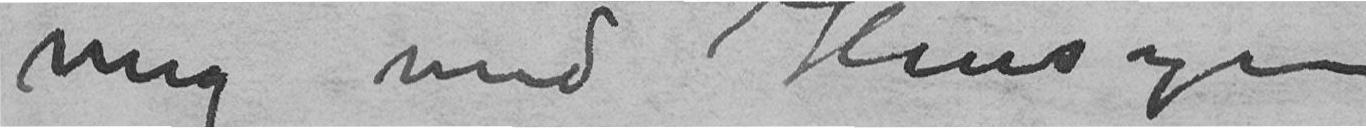mig med Hensyn
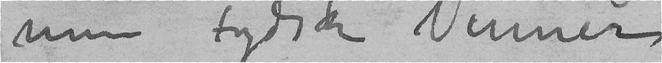mine tydske Venner
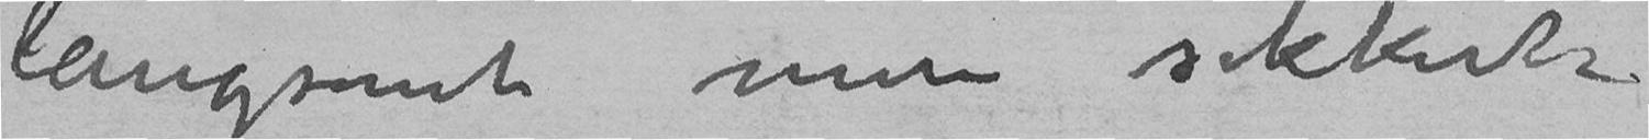langsomt men sikkert
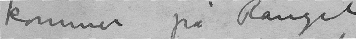kommer på Rangel
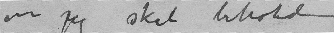om jeg skal beholde
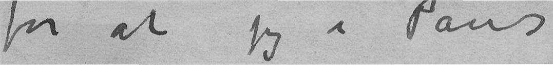for at jeg i Paris
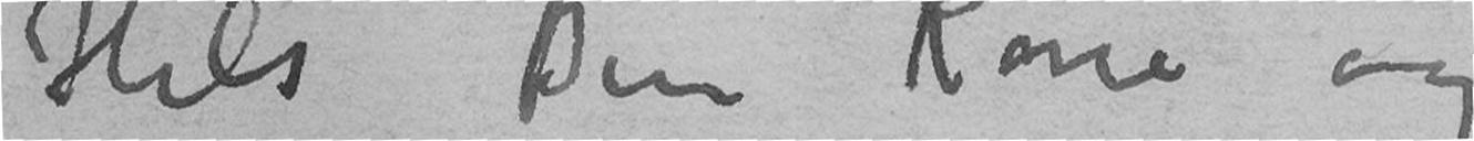Hils Din Kone og
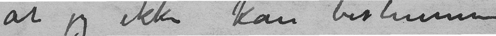at jeg ikke kan bestemme
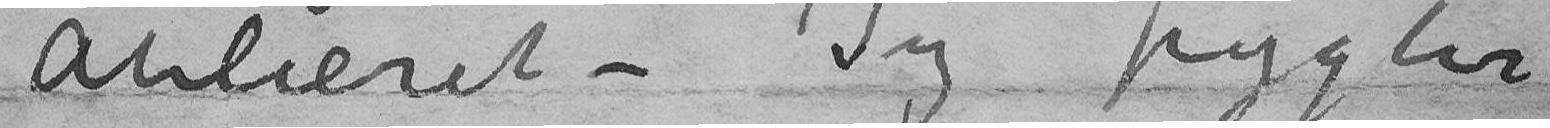Atelieret – Jeg frygter
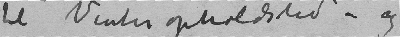til Vinteropholdsted – og
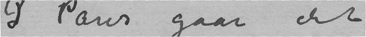I Paris gaar det
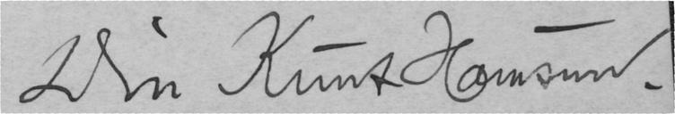Din Knut Hamsun.
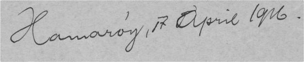Hamarøy, 17 April 1916.
